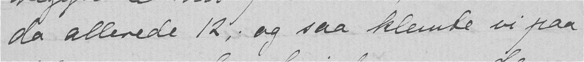da allerede 12, og saa klemte vi paa
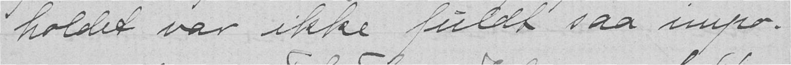holdet var ikke fuldt saa impo-
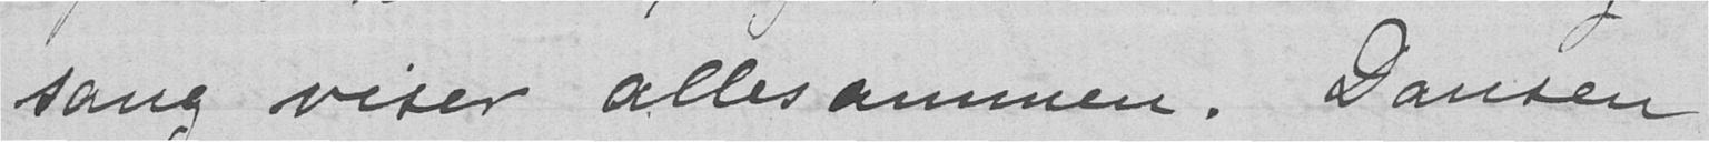sang viser allesammen. Dansen
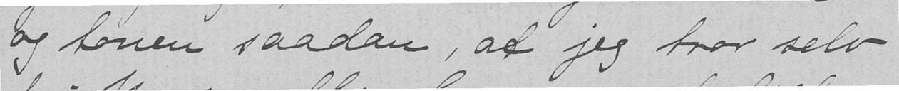og tonen saadan, at jeg tror selv
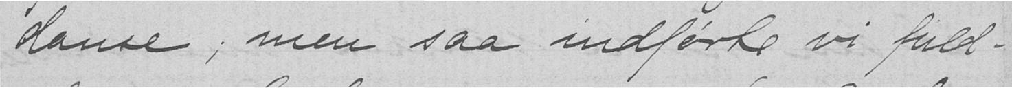danse; men saa indførte vi fuld-
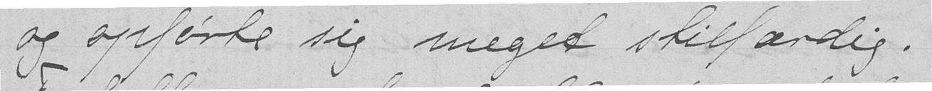og opførte sig meget stilfærdig.
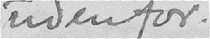udenfor.
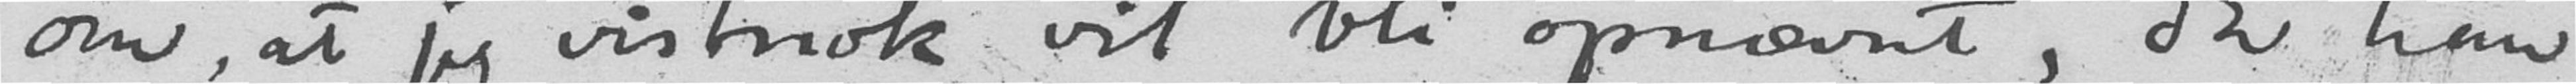om, at jeg vistnok vil bli opnævnt, da han
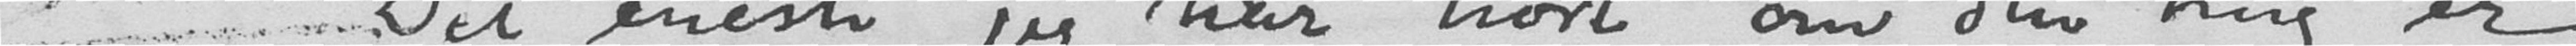Det eneste jeg har hørt om den ting er
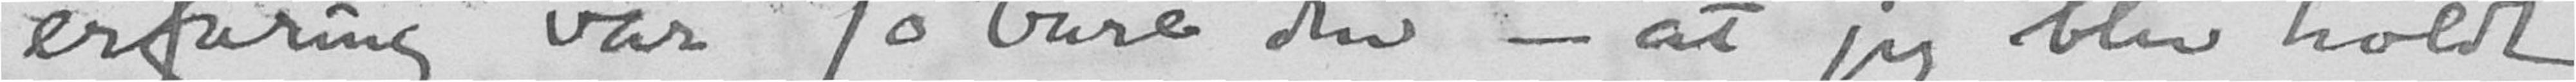erfaring var jo bare den - at jeg blev holdt
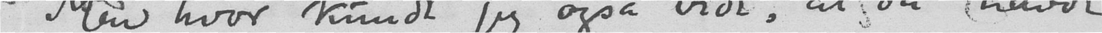Men hvor kunde jeg også vide, at du havde
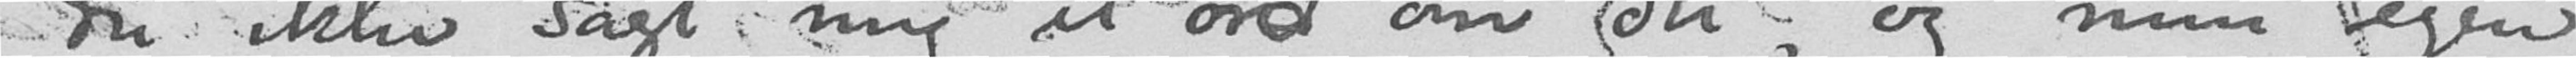du ikke sagt mig et ord om det, og min egen
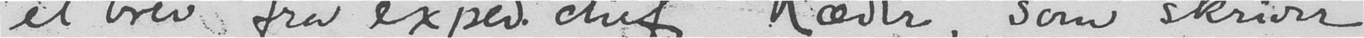et brev fra exped. chef Ræder, som skriver
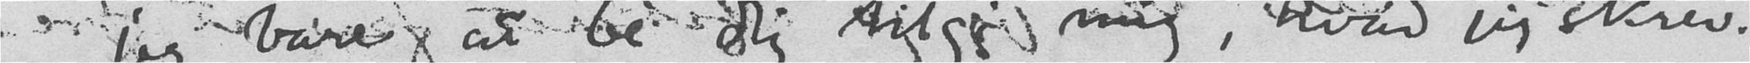jeg bare at be dig tilgi mig, hvad jeg skrev.
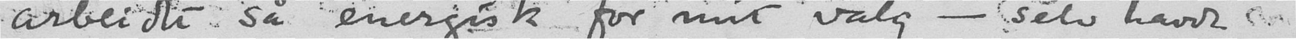arbeidet så energisk for mit valg - selv havde
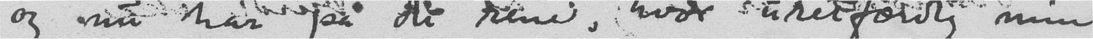og nu har på det rene, hvor uretfærdig min
